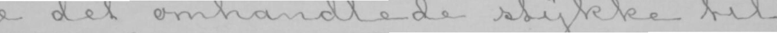ge det omhandlede stykke til
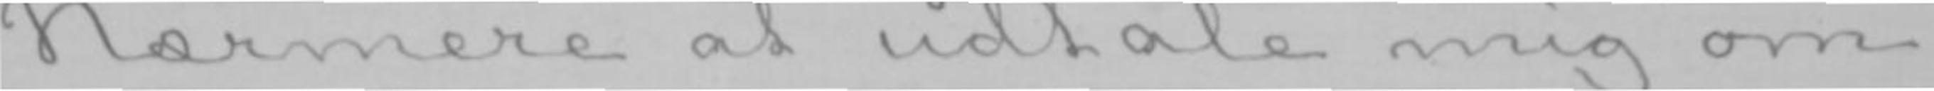Nærmere at udtale mig om
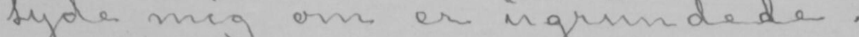tyde mig om er ugrundede.
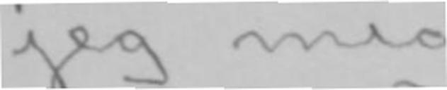jeg mig
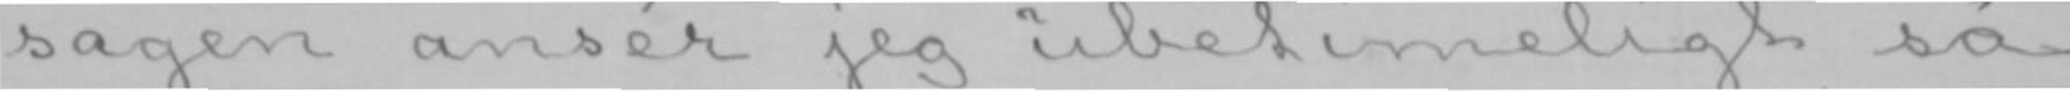sagen ansér jeg ubetimeligt så
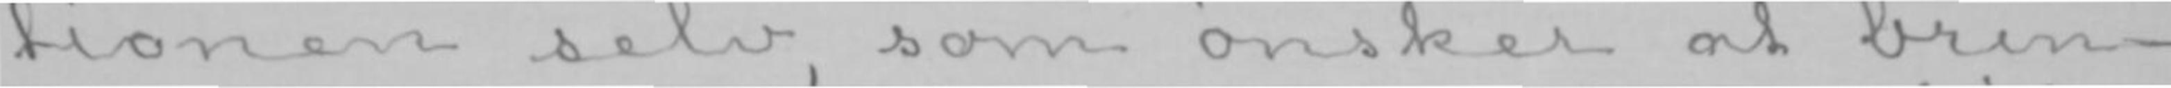tionen selv, som ønsker at brin-
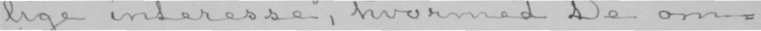lige interesse, hvormed De om-
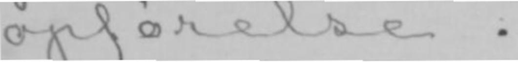opførelse.
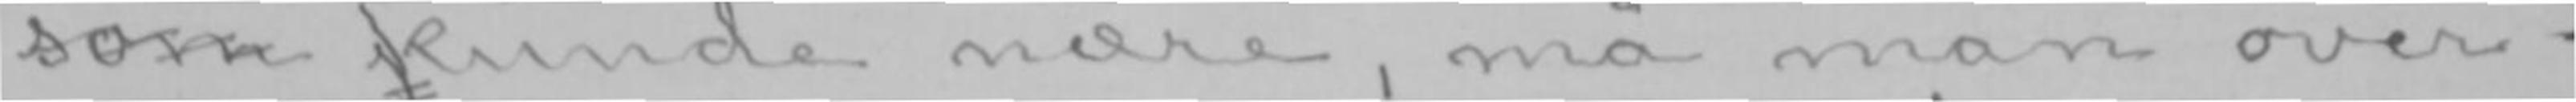som kunde nære, må man over-
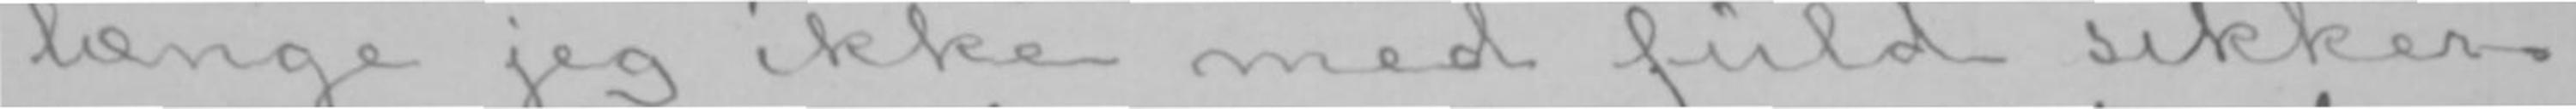længe jeg ikke med fuld sikker-
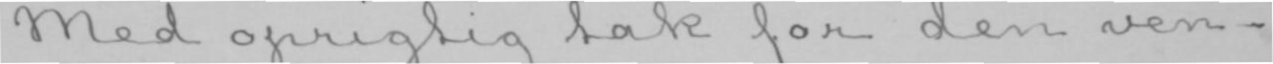Med oprigtig tak for den ven-
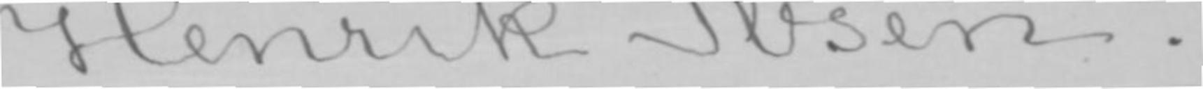Henrik Ibsen.
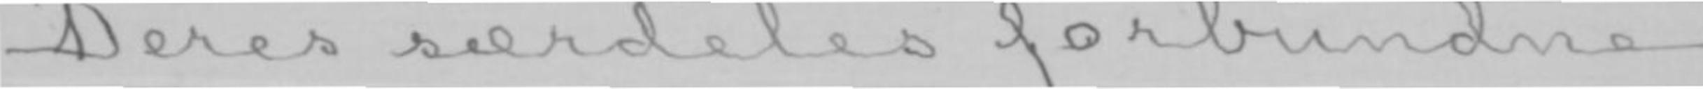Deres særdeles forbundne
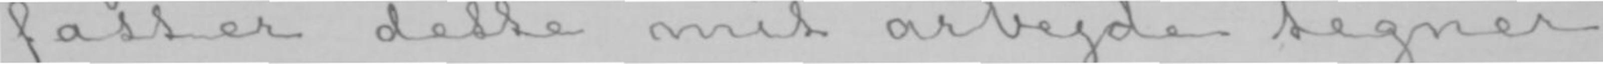fatter dette mit arbejde tegner
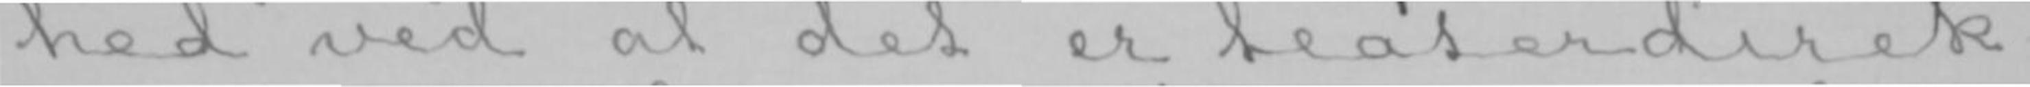hed ved at det er teaterdirek-
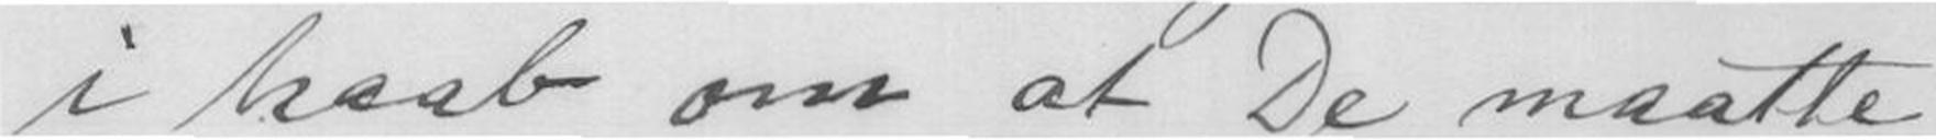i haab om at De maatte
